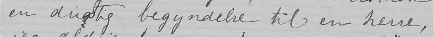en dristig begyndelse til en herre,
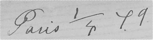Paris 1/4 79.
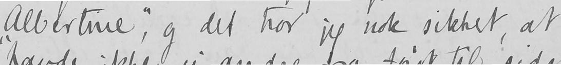"Albertine", og det tror jeg nok sikkert, at
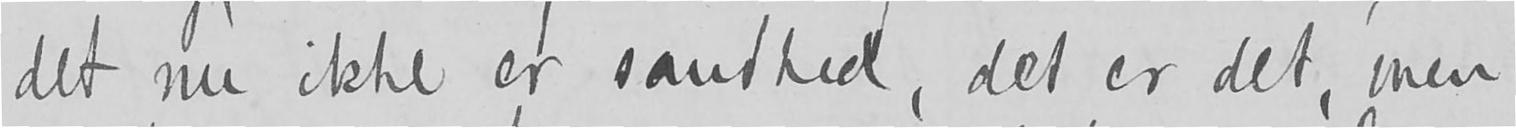det nu ikke er sandhed, det er det, men
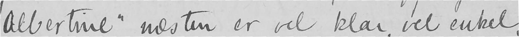"Albertine" næsten er vel klar, vel enkel,
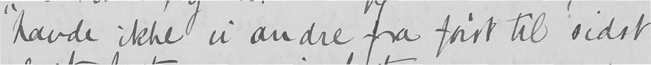havde ikke vi andre fra først til sidst
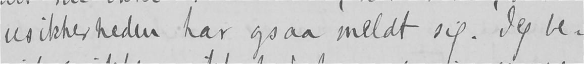usikkerheden har ogsaa meldt sig. Jeg be-
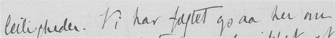leiligheder. Vi har fagtet ogsaa her om
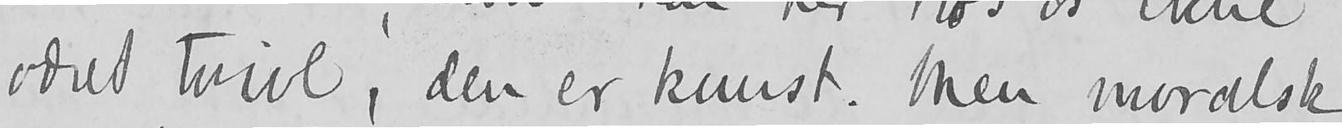været tvivl, den er kunst. Men moralsk
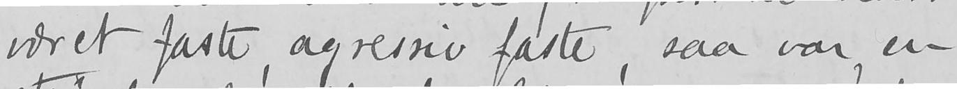været faste, agressiv faste, saa var en
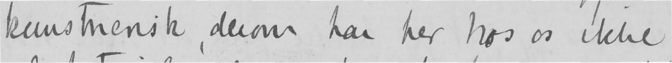kunstnerisk, derom har her hos os ikke
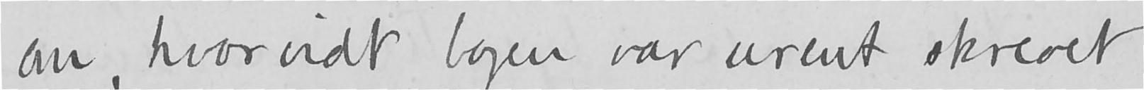om, hvorvidt bogen var urent skrevet
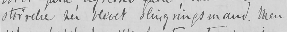størrelse her blevet slingringsmand. Men
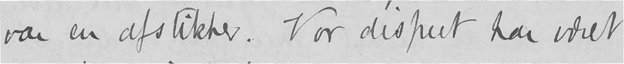var en afstikker. Vor disput har været
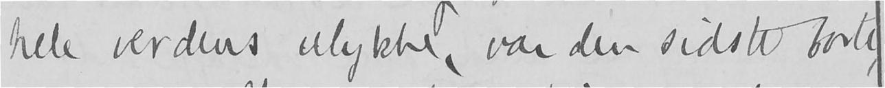hele verdens ulykke, var den sidste borte,
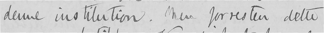denne institution. Men forresten dette
